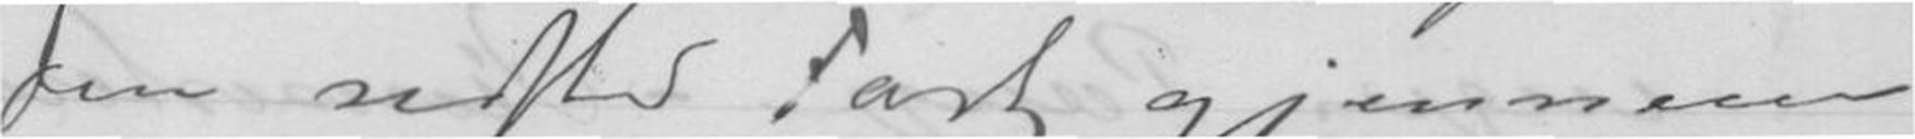den sidste Fart gjennem
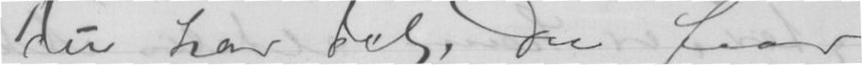du har det, Du faar
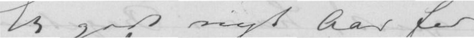Et godt nyt Aar for
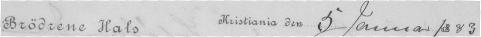Brødrene Hals Kristiania den 5 Januar 1883
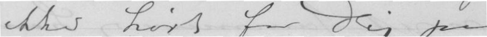ikke hørt fra Dig paa
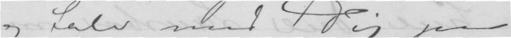og tale med Dig paa
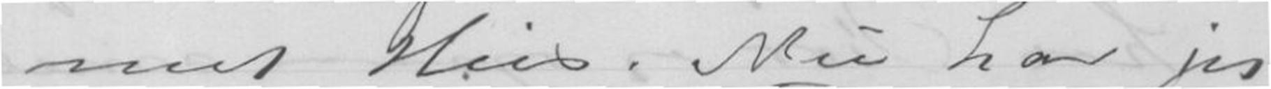mit Hus. Nu har jeg
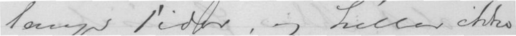lange Tider, og heller ikke
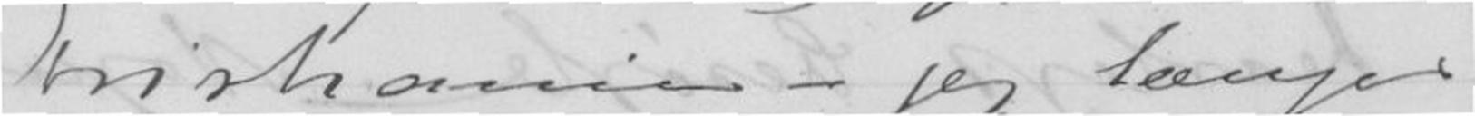Kristiania - jeg længes
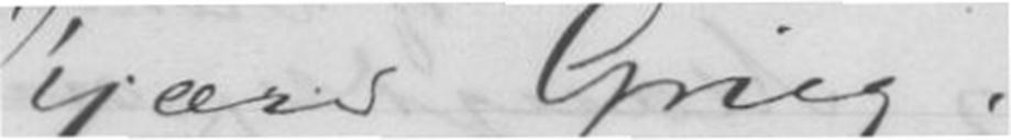Kjære Grieg!
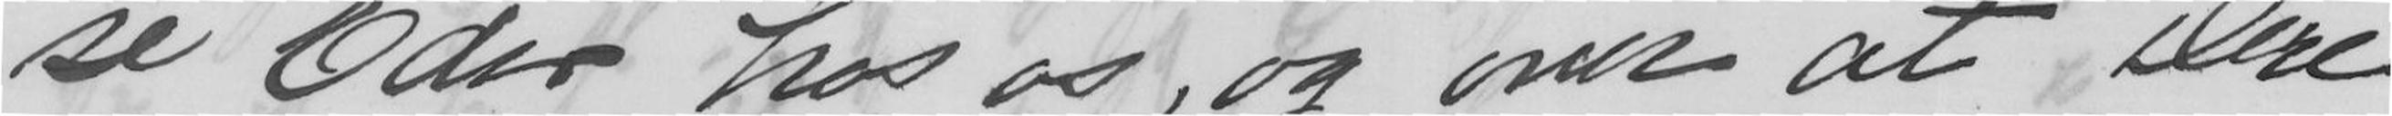se Eder hos os, og over at Dere
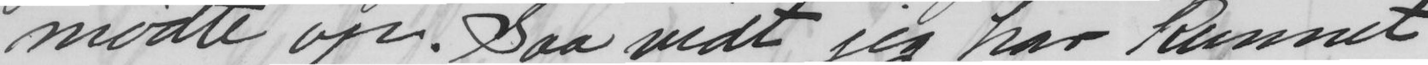mødte op. Saa vidt jeg har kunnet
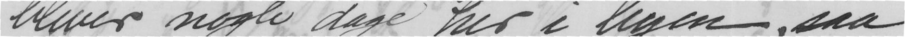bliver nogle dage her i byen, saa
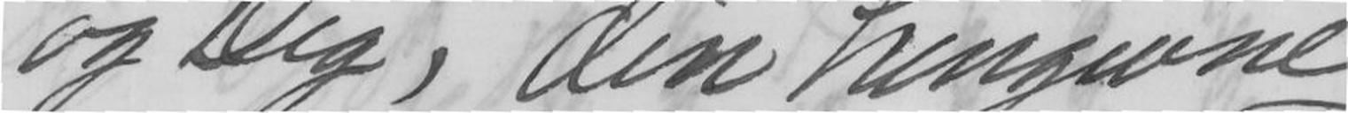og Dig, din hengivne
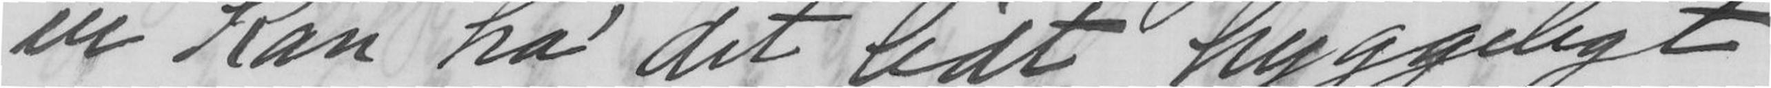vi kan ha' det lidt hyggeligt
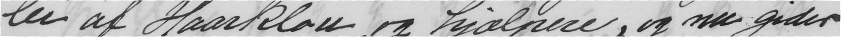lei af Haarklou og hjælpere, og nu gider
